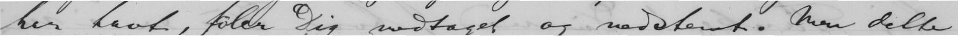har havt, føler Dig medtaget og nedstemt. Men dette
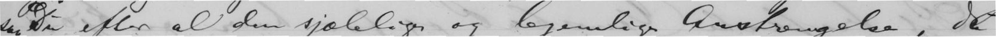saa Du efter al den sjælelige og legemlige Anstrengelse, Du
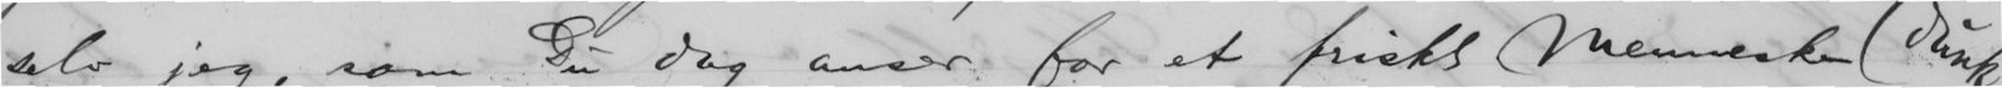selv jeg, som Du dog anser for et friskt Menneske (dunk
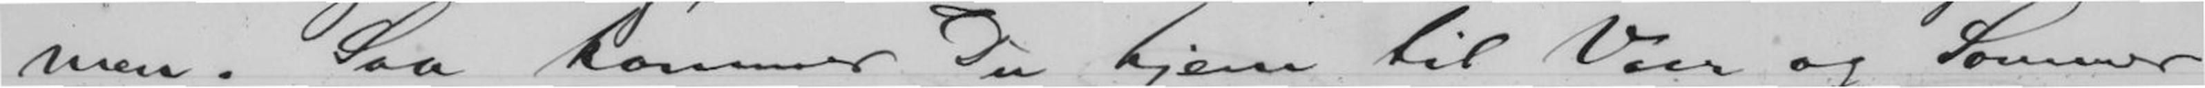men. Saa kommer Du hjem til Vaar og Sommer
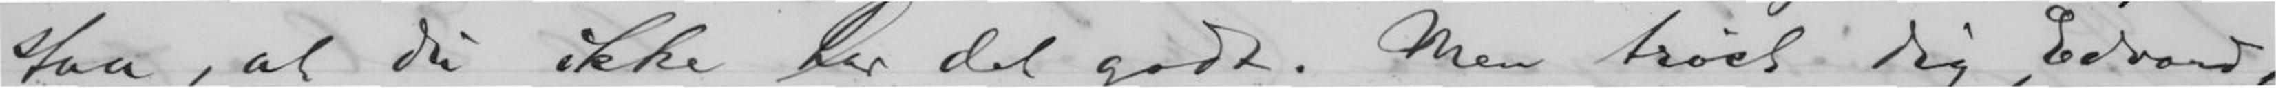staa, at Du ikke har det godt. Men trøst Dig, Edvard,
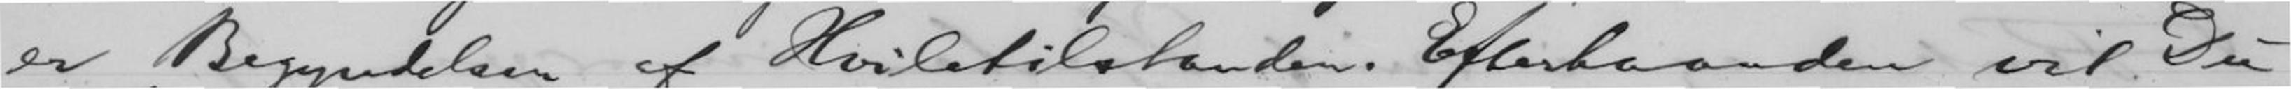er Begyndelsen af Hviletilstanden. Efterhaanden vil Du
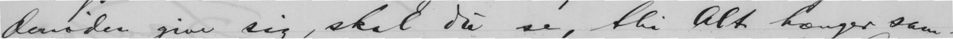denøden give sig, skal du se, thi Alt hænger sam-
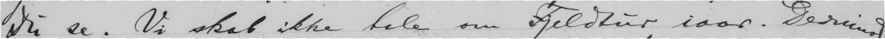Du se. Vi skal ikke tale om Fjeldtur iaar. Derimod
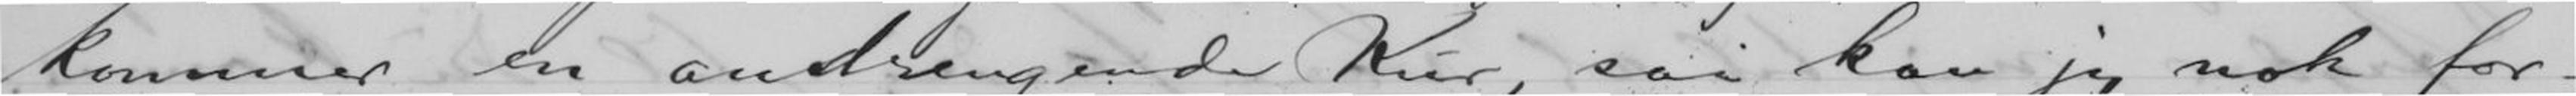kommer en anstrengende Kur, saa kan jeg nok for-
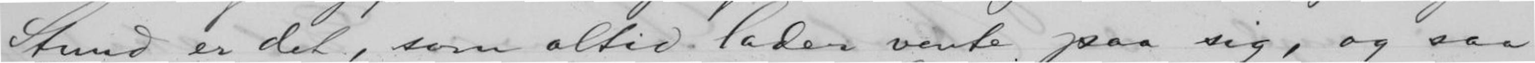Stund er det, som altid lader vente paa sig, og saa
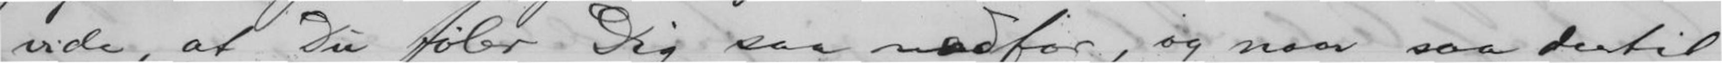vide, at Du føler Dig saa nedfor, og naar saa dertil
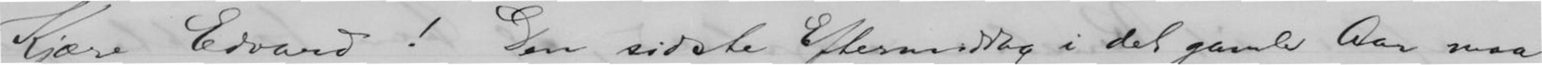Kjære Edvard! Den sidste Eftermiddag i det gamle Aar maa
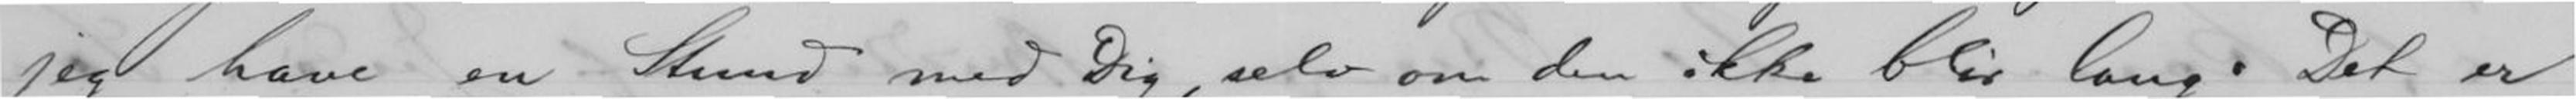jeg have en Stund med Dig, selv om den ikke blir lang. Det er
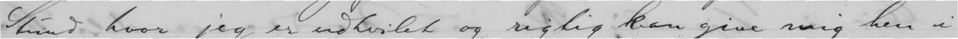Stund hvor jeg er udhvilet og rigtig kan give mig hen i
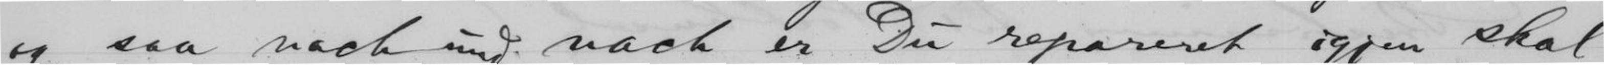og saa nach und nach er Du repareret igjen skal
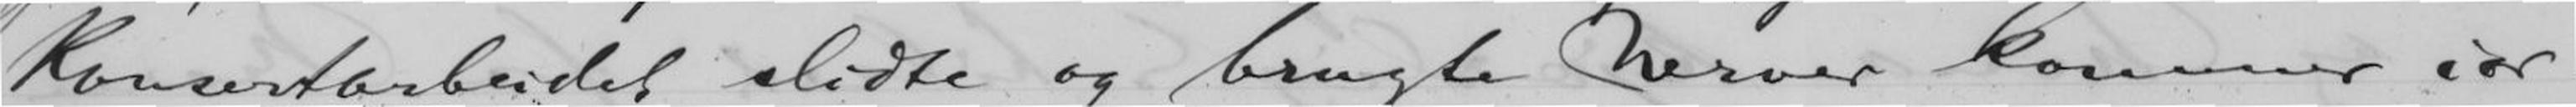Konsertarbeidet slidte og Brugte Nerver kommer ior-
